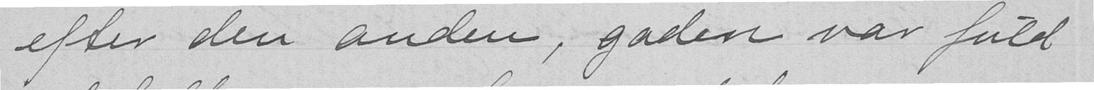efter den anden, gaden var fuld
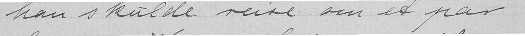han skulde reise om et par
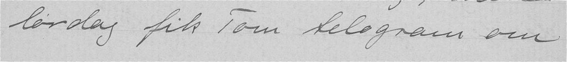lørdag fik Tom telegram om
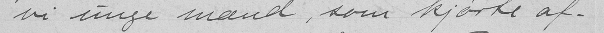vi unge mænd, som kjørte af-
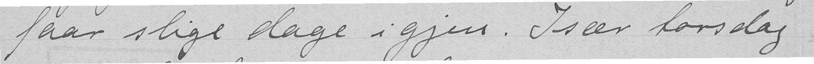faar slige dage igjen. Især torsdag
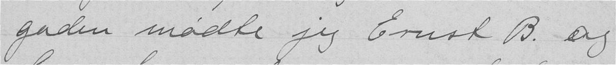gaden mødte jeg Ernst B. og
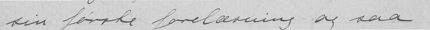sin første forelæsning og saa
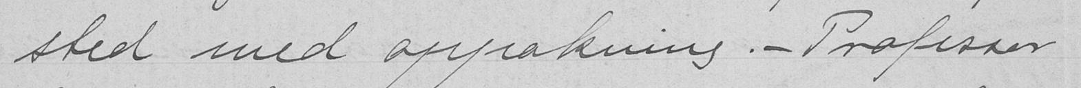sted med oppakning. - Professor
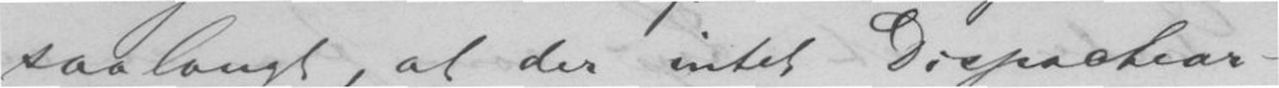saa langt, at der intet Dispachear-
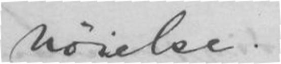nøielse. -
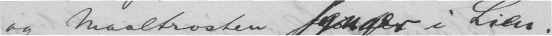og Maaltrosten synger i Lien.
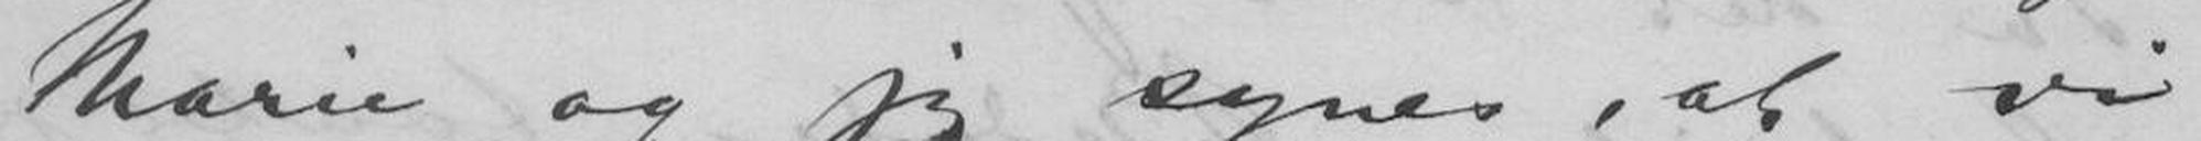Marie og jeg synes, at vi
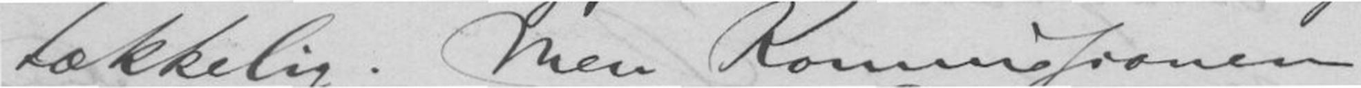tækkelig. Men Kommessionen
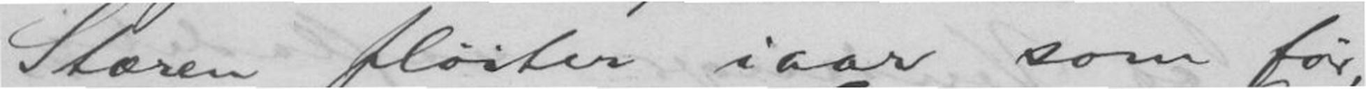Stæren fløiter iaar som før,
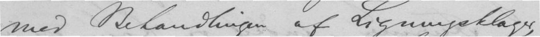med Behandlingen af Ligningsklager,
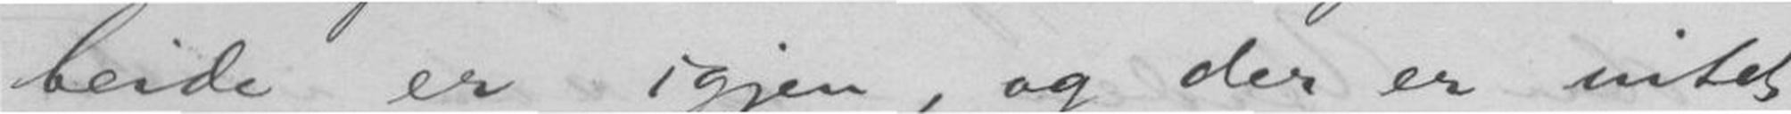beide er igjen, og der er intet
